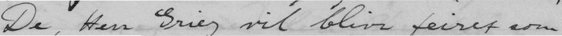De, Herr Grieg vil blive feiret som
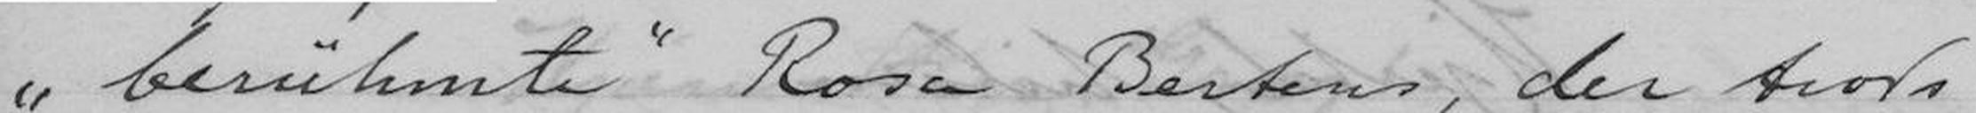"berühmte" Rosa Bertens, der trods
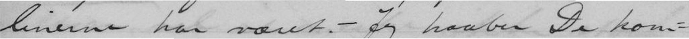linerne har været. - Jeg haaber De kom-
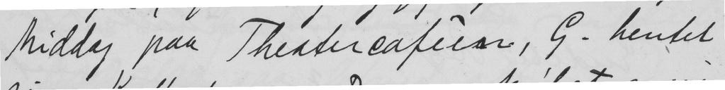Middag paa Theatercafeén, G. hentet
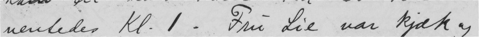ventedes Kl. 1. Fru Lie var kjæk og
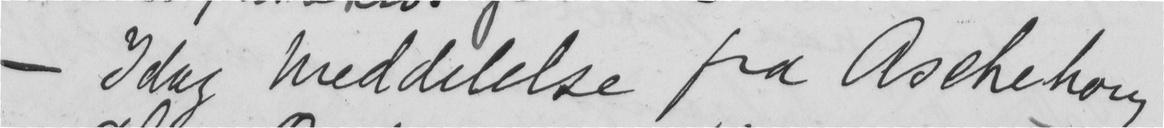- Idag Meddelelse fra Aschehoug
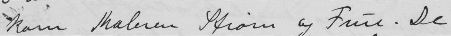kom Maleren Strøm og Frue. De
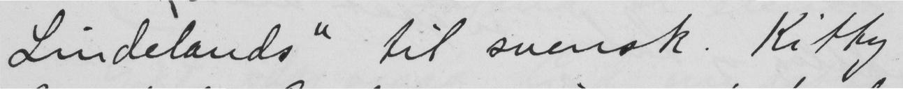Lindelands" til svensk. Kitty
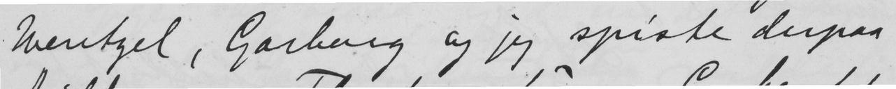Wentzel, Garbong og jeg spiste derpaa
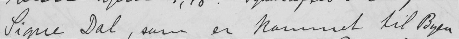Signe Dal, som er kommet til Byen
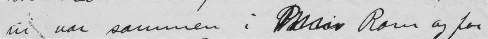vi var sammen i  Rom og for
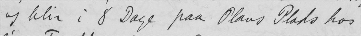og blir i 8 Dage paa Olavs Plads hos
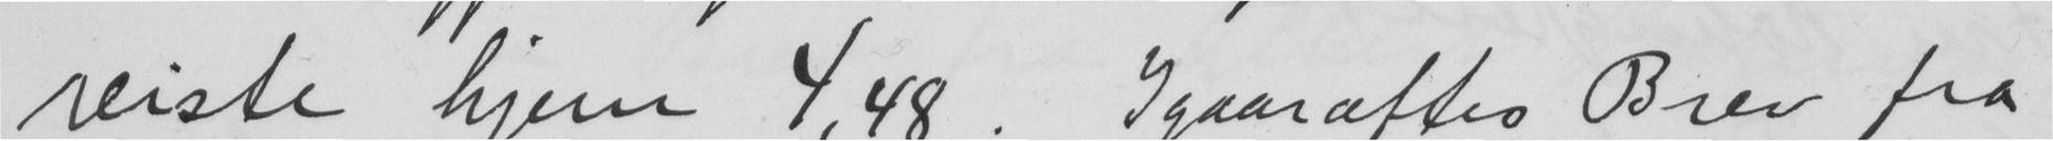reiste hjem 4,48. Igaaraftes Brev fra
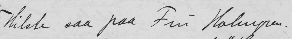Hilste saa paa Fiu Holmgren.
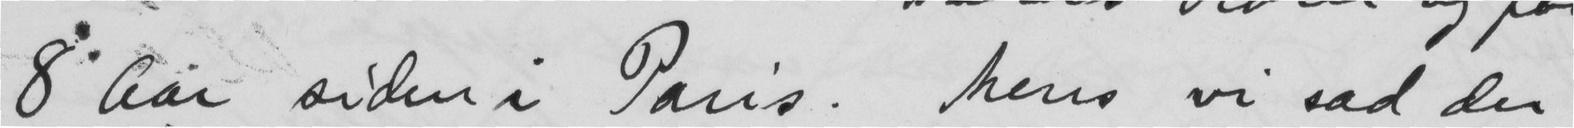8 Aar siden i Paris. Mens vi sad der
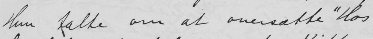Hun talte om at oversætte "Hos
